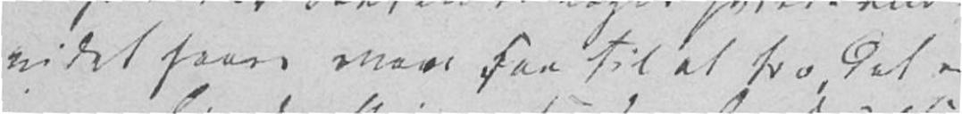videt faaer man her til at tro det er
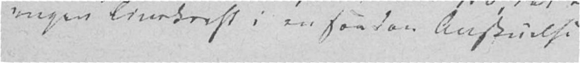ingen Livskraft i en saadan Anskuelse
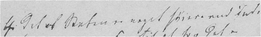thi Det at Staten er noget høiere end Indi-
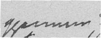gjennem
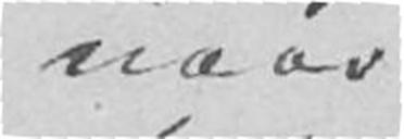naar
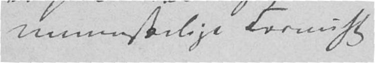menneskelige Fornuft
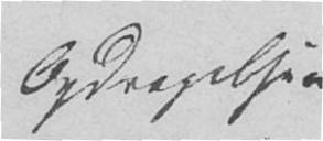Opdragelsen
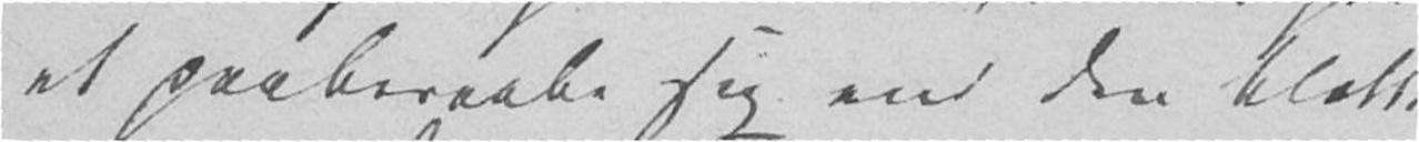at paaberaabe sig end den blotte
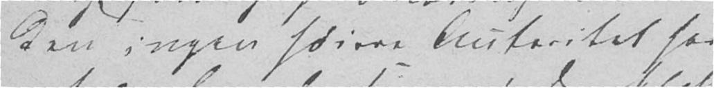den ingen høiere Autoritet har
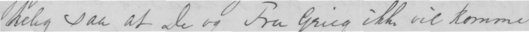kelig saa at De og Fru Grieg ikke vil komme
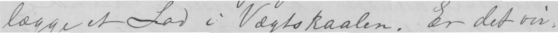lægge et Lod i Vegtskaalen. Er det vir-
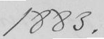1883.
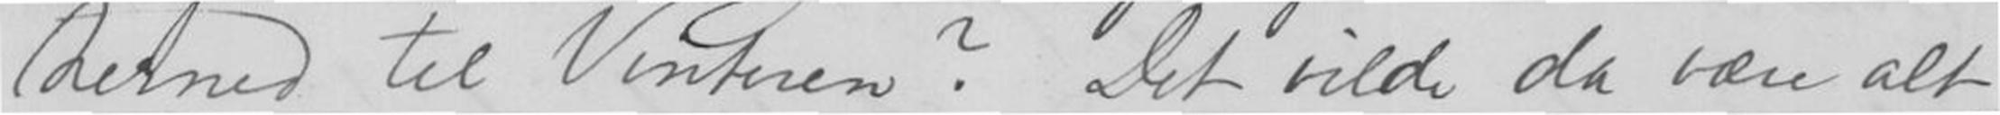herned til Vinteren? Det vilde da være alt-
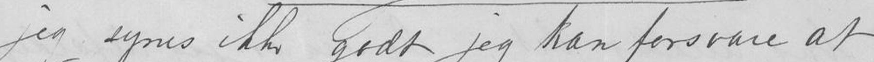jeg synes ikke godt jeg kan forsvare at
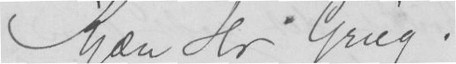Kjære Hr. Grieg!
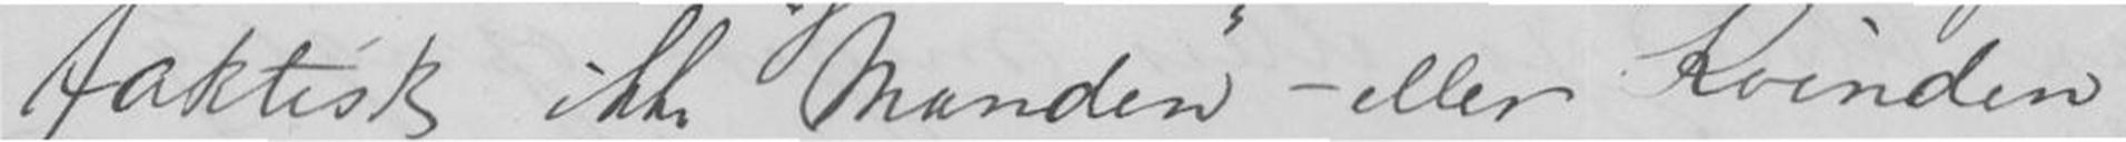faktisk ikke "Manden" - eller Kvinden,
